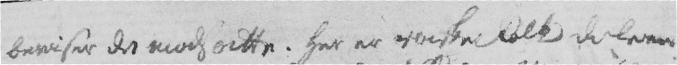beviser det modsatte. Her er raske Folk der lever
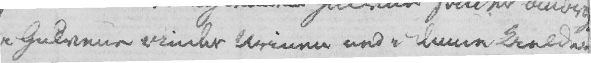i Gulvene rinder Urinen ned i denne Kielder
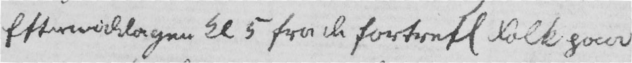Eftermiddagen Kl 5 fra de fortrefl Folk paa
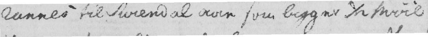Rannes til Surendal aae som ligger 1/2 Miil
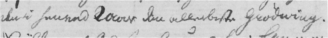den i henved 2 Aar den allerbeste Giødning.
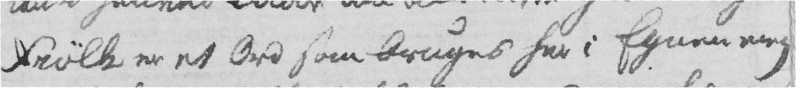Fiølk er et Ord som bruges her i Egnen nmg
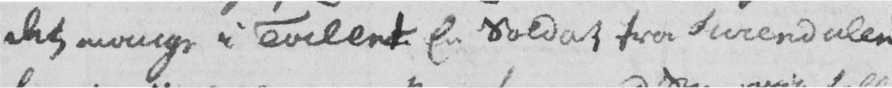det mange i Tallet. En Soldat fra Surendalen
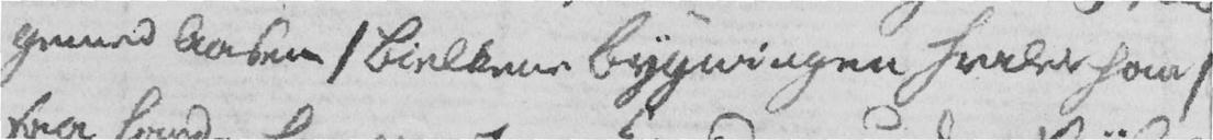gemed Aasen / Bielkene Bygningen hviler paa /
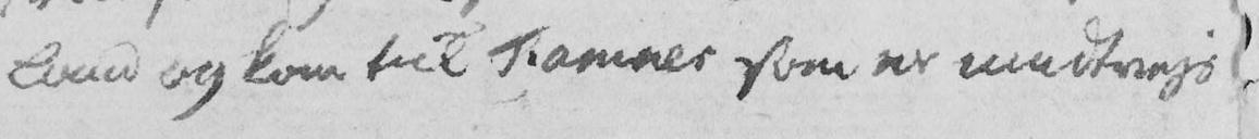Land og kom til Framnes som er midtvejs
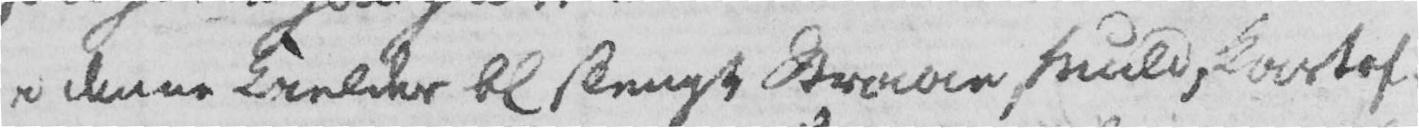i denne Kielder bl slengt Straae, Muld, Kostaf-
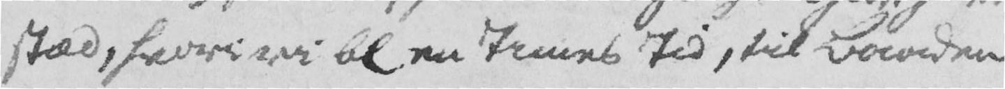stæd, hvori vi bl en Times Tid, til Baaden
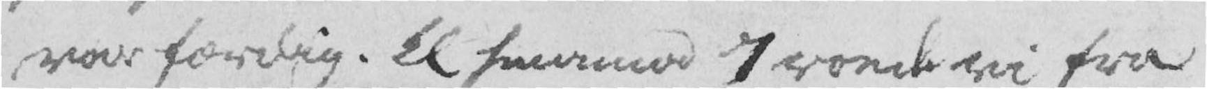var færdig. Kl henimod 7 roede vi fra
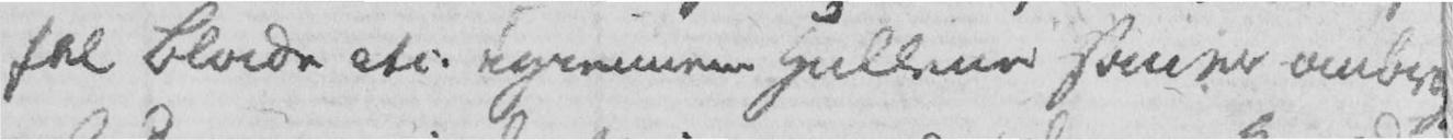fal Blade etc. igiennem Hullene som er anbragte
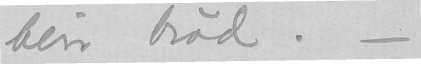blir brød. -
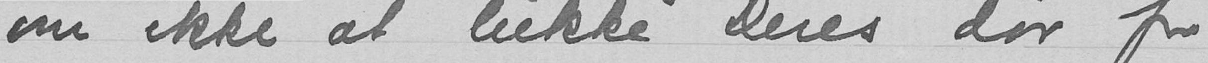om ikke at lukke Deres dør for
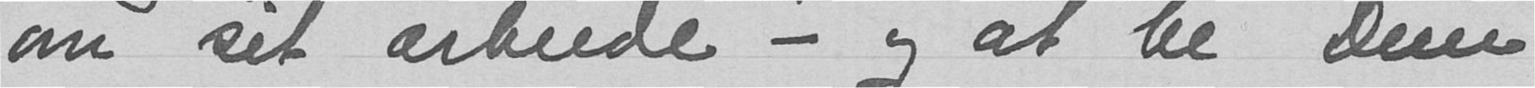om sit arbeide - og at be Dem
